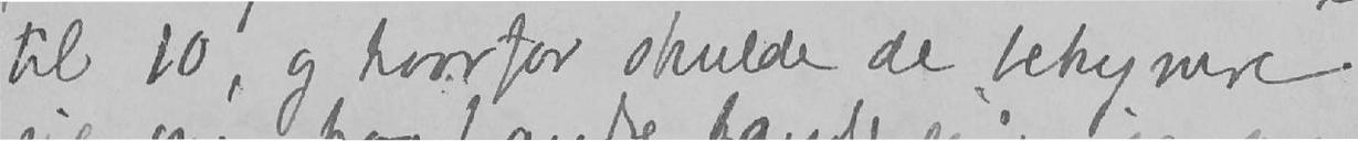til 10, og hvorfor skulde de bekymre
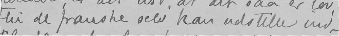thi de franske selv kan udstille ind-
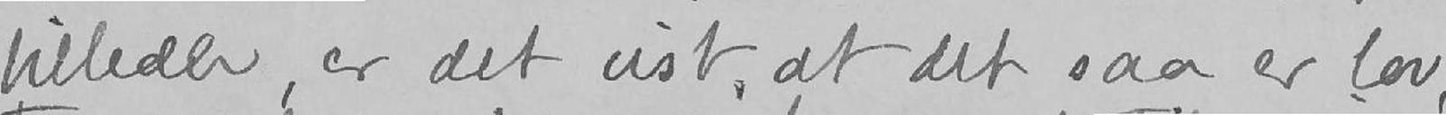billeder, er det vist, at det saa er lov,
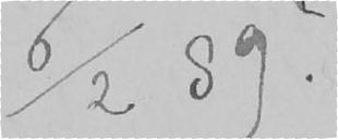6/2 89.
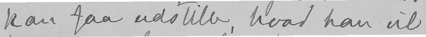kan faa udstille, hvad han vil,
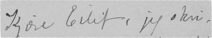Kjære Eilif, jeg skri-
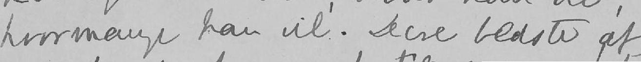hvormange han vil. Dere bedste af
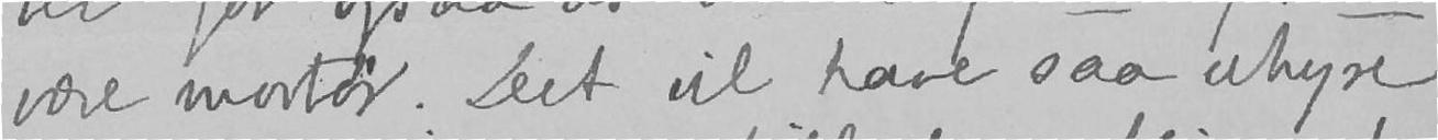være montør. Det vil have saa uhyre
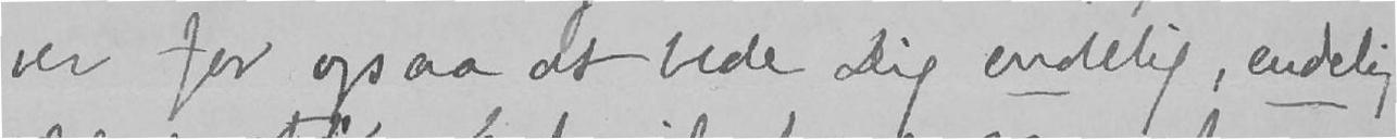ver for ogsaa at bede Dig endelig, endelig
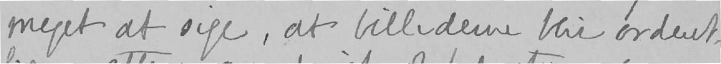meget at sige, at billederne blir ordent-
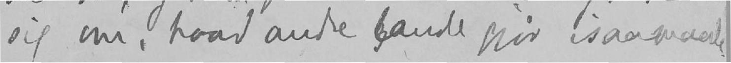sig om, hvad andre lande gjør isaamaade.
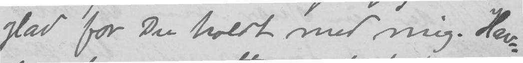glad for Du holdt med mig. Hav-
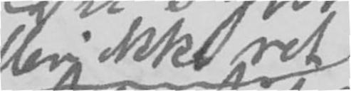eller ikke ret
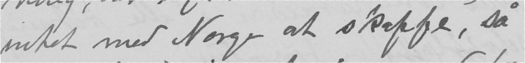intet med Norge at skaffe, så
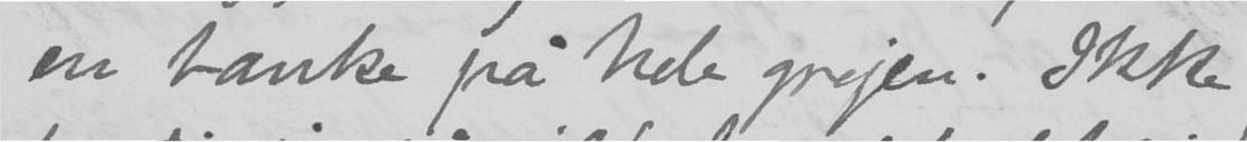en tanke på hele grejen. Ikke
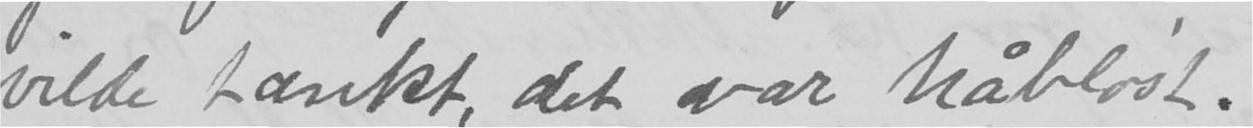vilde tænkt, det var håbløst.
